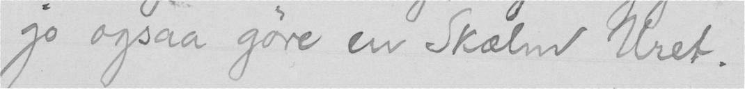jo ogsaa gøre en Skælm Uret.
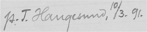p.T. Haugesund, 10/3. 91.
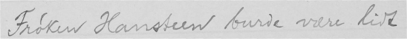Frøken Hansteen burde være lidt
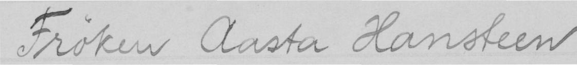Frøken Aasta Hansteen
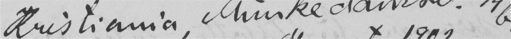Kristiania, Munkedamsv. 44 b.,
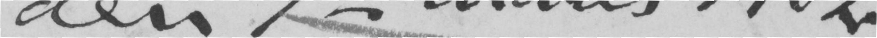den 9de marts 1902.
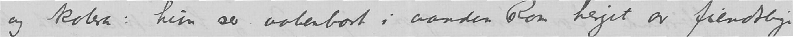og kolera: hun ser aabenbart i aanden Rom herjet av fiendtlige
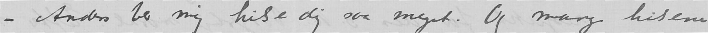Anders ber mig hilse dig saa meget. Og mange hilsener
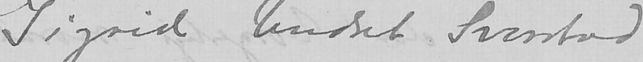Sigrid Undset Svarstad
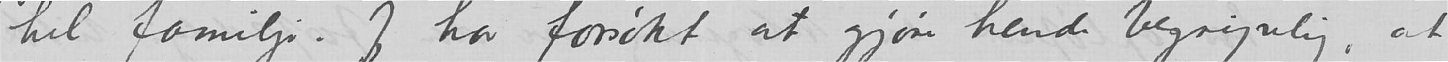hel familje. Jeg har forsøkt at gjøre hende begripelig, at
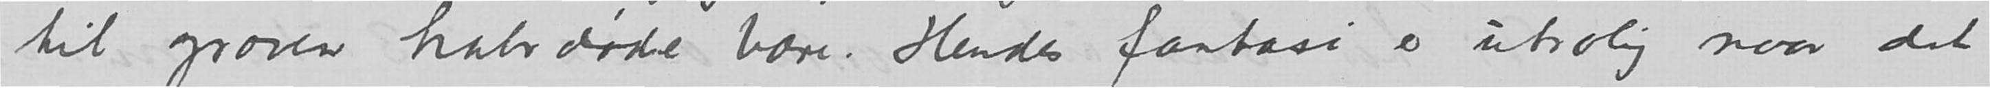til graven halvdøde bare. Hendes fantasi er utrolig naar
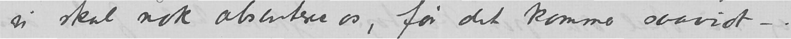vi skal nok absentere os, før det kommer saavidt -.
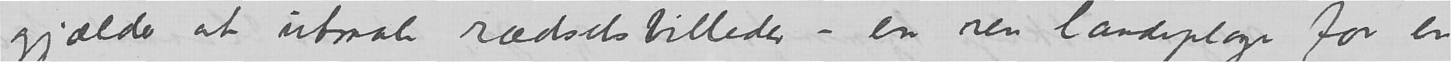det gjælder at utmale rædselsbilleder - en ren landeplage for en
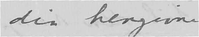din hengivne
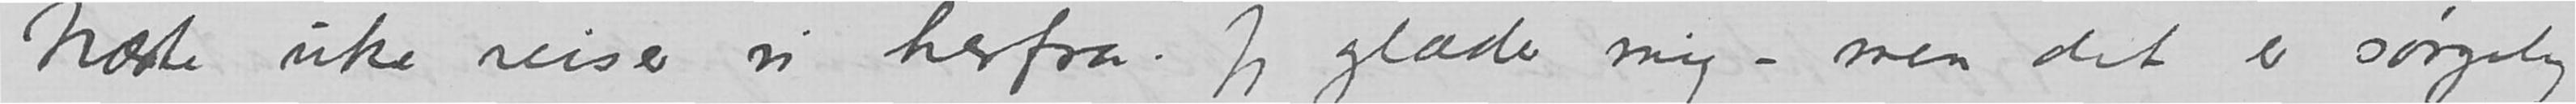Næste uke reiser vi herfra. Jeg glæder mig - men det er sørgelig
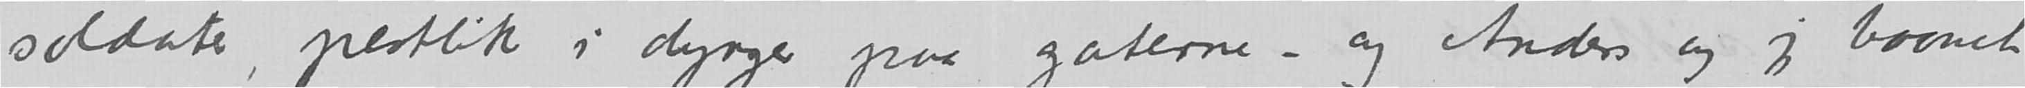soldater, pestlik i dynger paa gaterne - og Anders og jeg baaret
